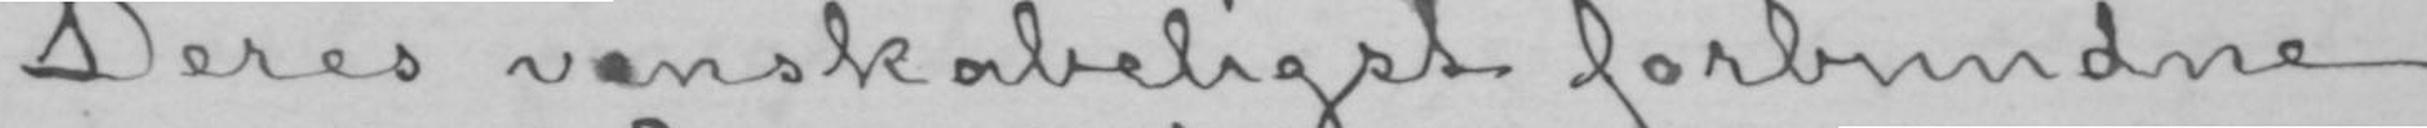Deres venskabeligst forbundne
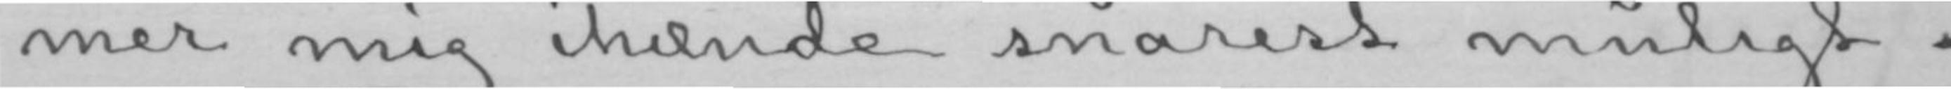mer mig ihænde snarest muligt.
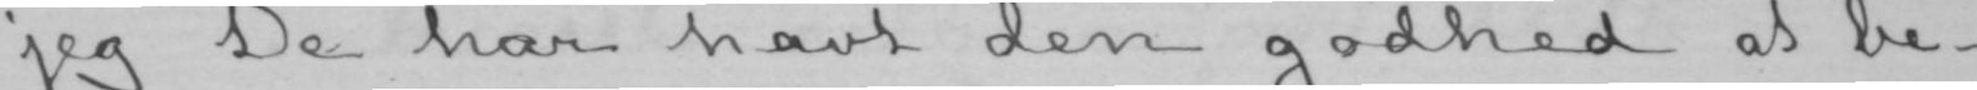jeg De har havt den godhed at be-
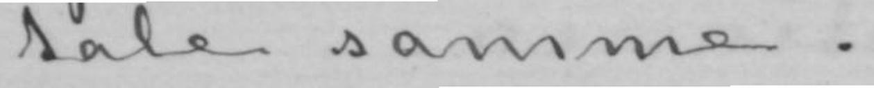tale samme.
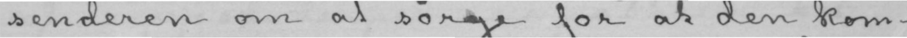senderen om at sørge for at den kom-
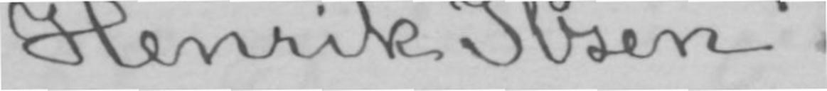Henrik Ibsen.
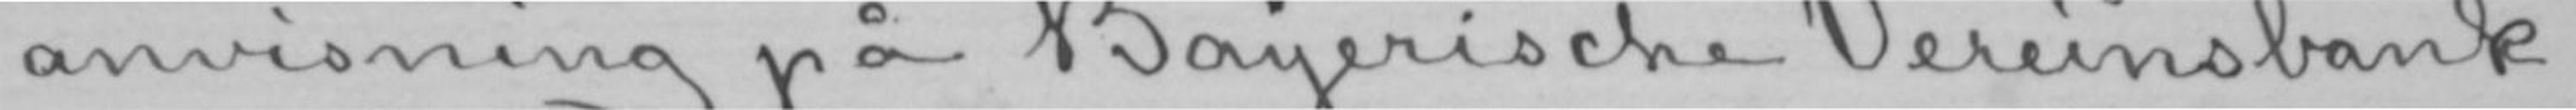anvisning på Bayerische Vereinsbank.
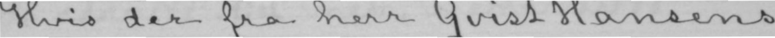Hvis der fra herr Qvist Hansens
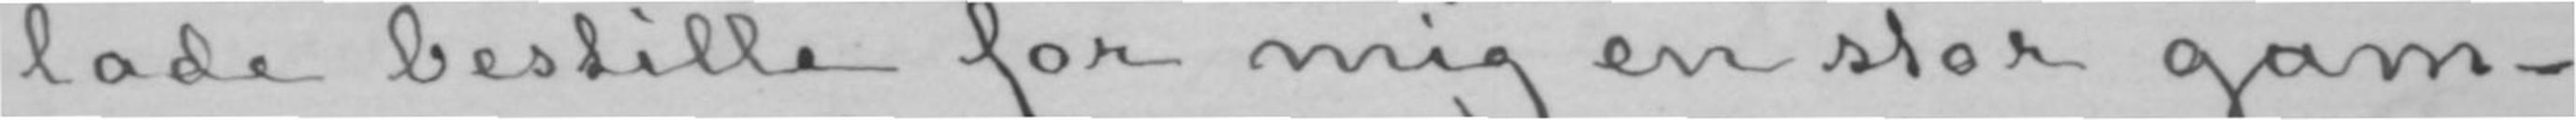lade bestille for mig en stor gam-
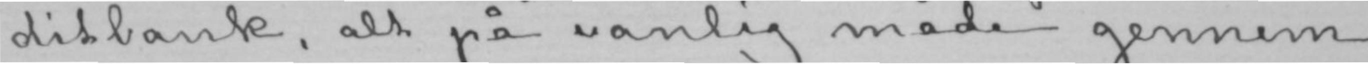ditbank, alt på vanlig måde gennem
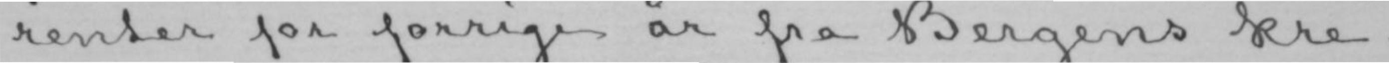renter for forrige år fra Bergens kre-
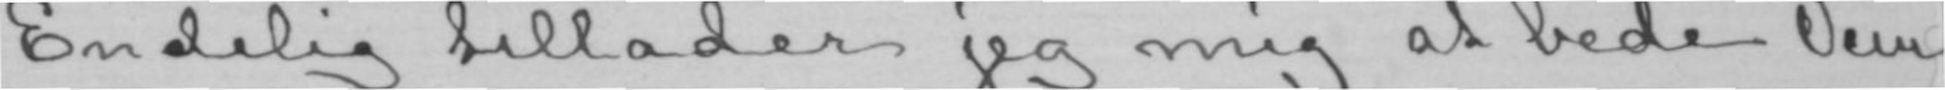Endelig tillader jeg mig at bede Dem
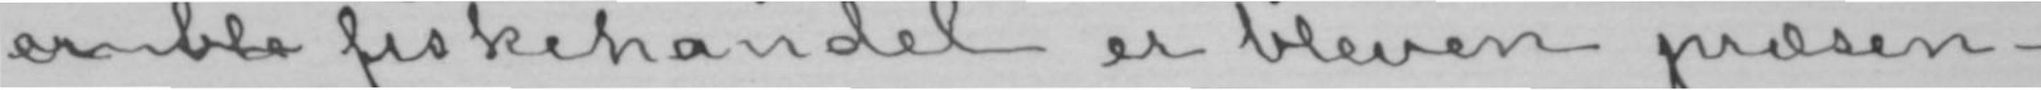er ble fiskehandel er bleven præsen-
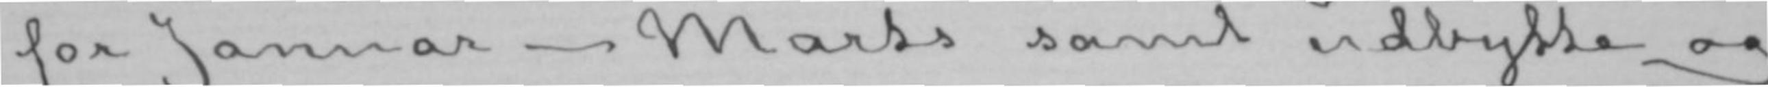for Januar-Marts samt udbytte og
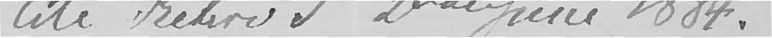Cité Retiro 3 2den Juni 1884.
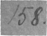158.
